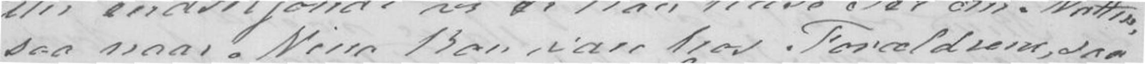saa naar Nina kan være hos Forældrede, saa
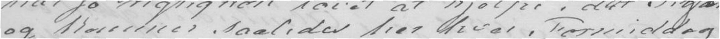og kommer saaledes her hver Formiddag
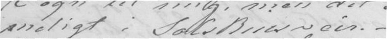meligt i Solskinsveir. -
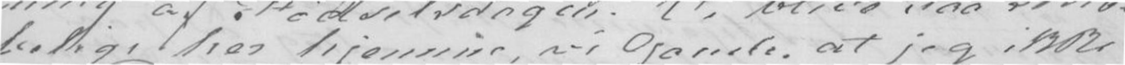belige her hjemme, vi gamle, at jeg ikke
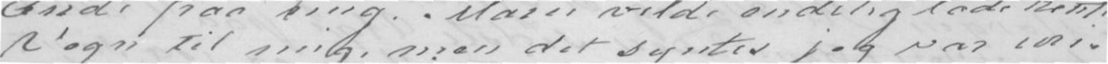Vogn til mig, men det syntes jeg var uri-
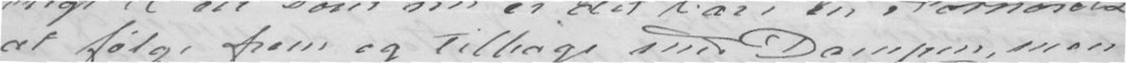at følge frem og tilbage med Dampen, men
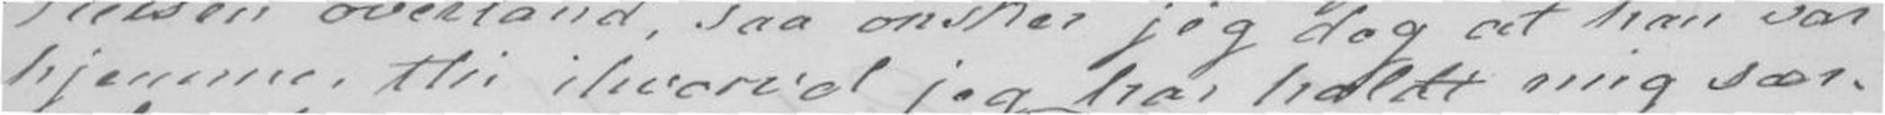hjemme, thi ihvorvel jeg har holdt mig sær-
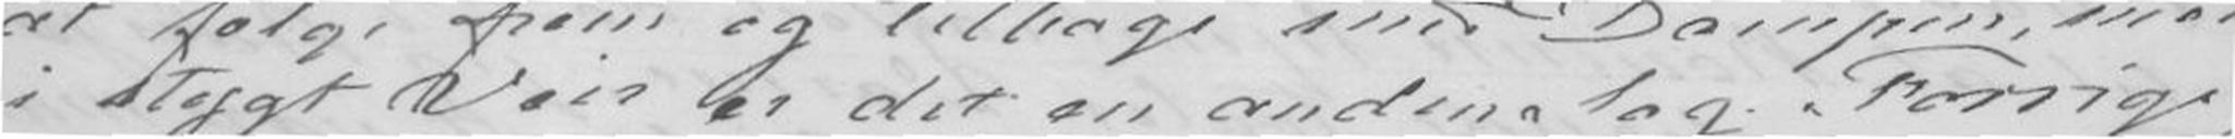i stygt Veir er det en anden Sag. Forrige
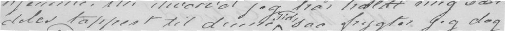deles tappert til denne Tid saa frygter jeg dog
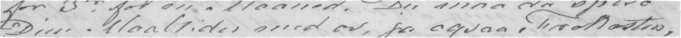Dine Maaltider med os, ja ogsaa Frokosten,
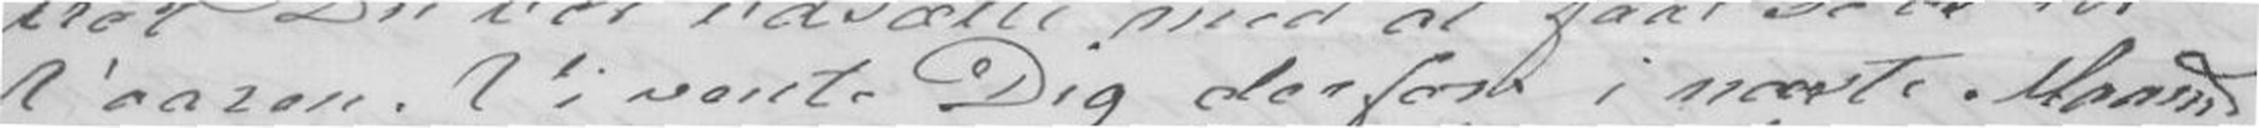Vaaren. Vi vente Dig derfor i næste Maaned,
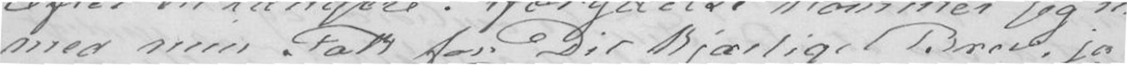med Tak for Dit kjærlige Brev, ja
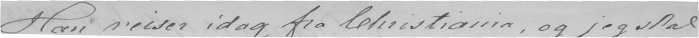Han reiser idag fra Christiania, og jeg skal
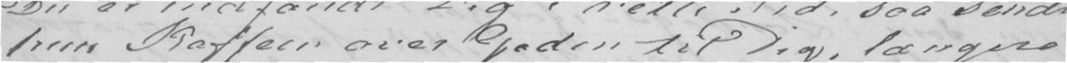hun Kaffen over Gaden til Dig, længere
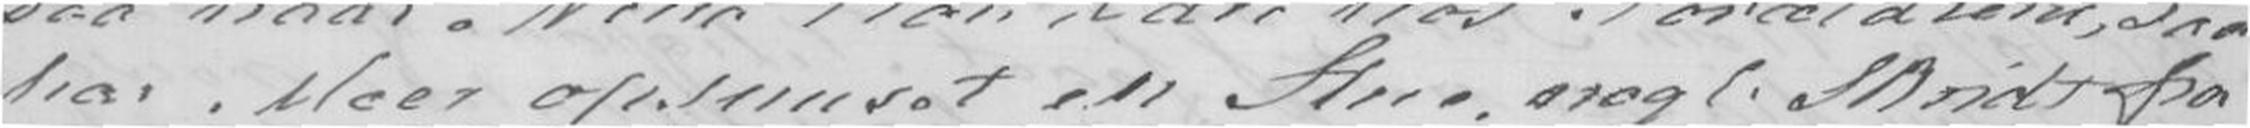har Moer opsnuset en Stue, nogle Skridt fra
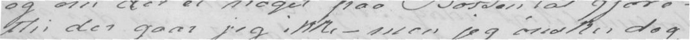thi der gaar jeg ikke - men jeg ønsker dog
